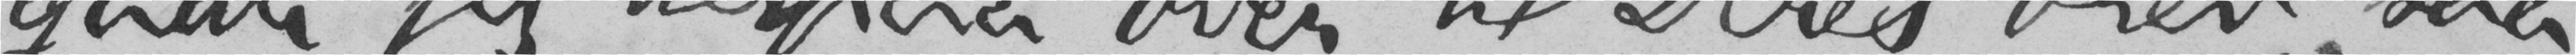Gaar jeg derpaa over til Deres brev, saa
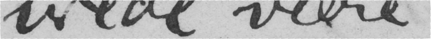vilde være
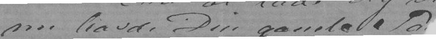nu havde Din gamle Fa
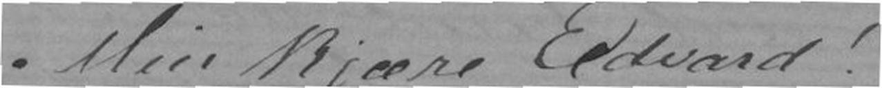Min kjære Edvard!
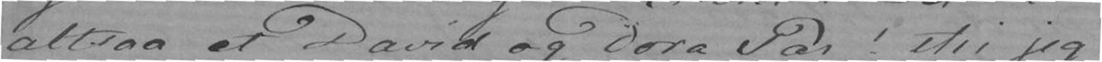altsaa et David og Dora Par! thi jeg
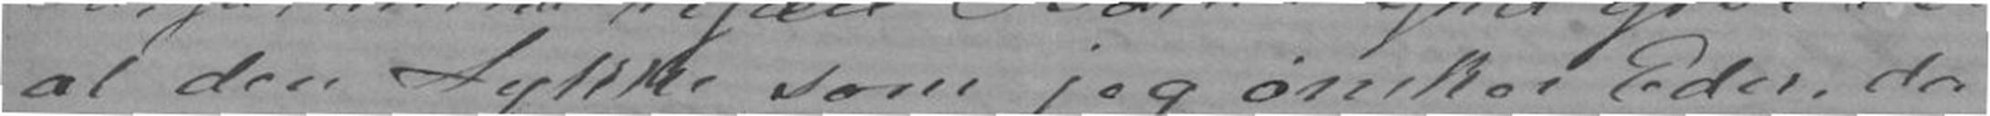al den Lykke som jeg ønsker Eder, da
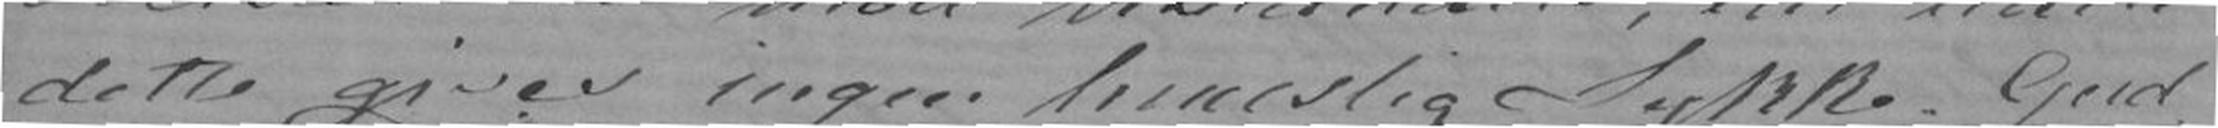dette gives ingen huuslig Lykke. Gud
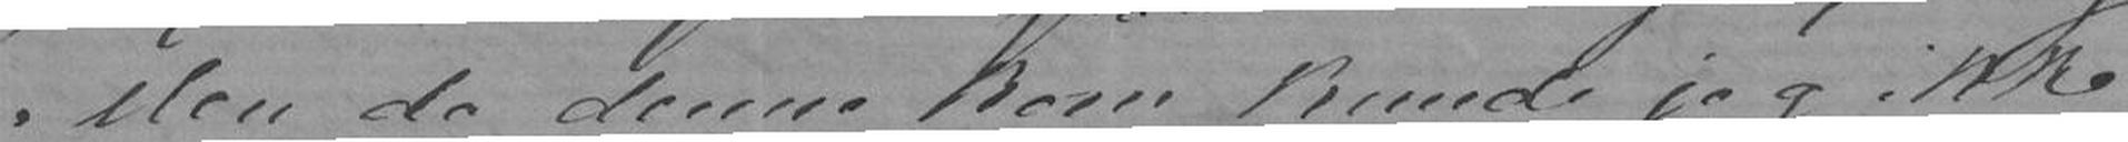Men da denne kom kunde jeg ikke
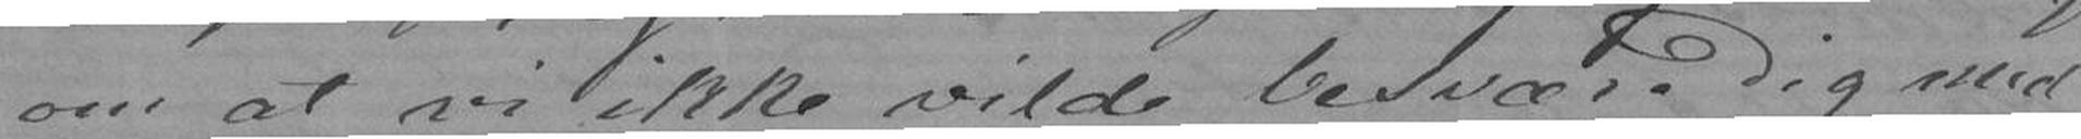om at vi ikke vilde besvære Dig med
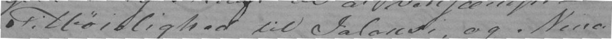Tilbøielighed til Jalousi, og Nina
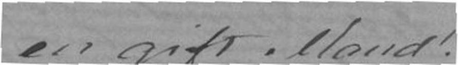Saa er Du da nu en gift Mand!
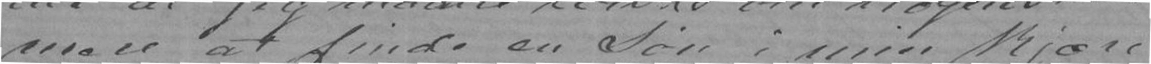mere at finde en Søn i min kjære
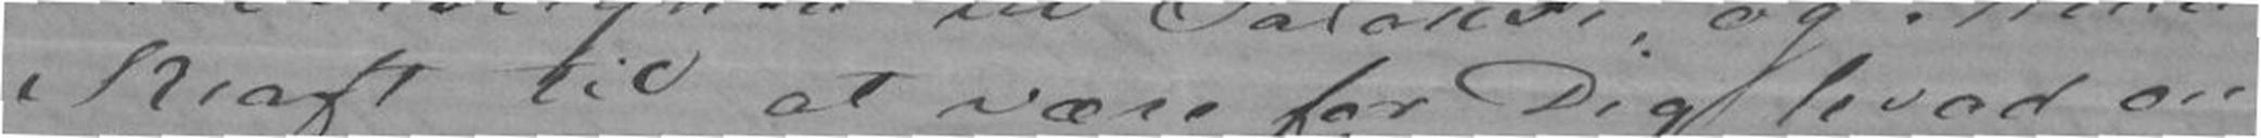Kraft til at være for Dig hvad en
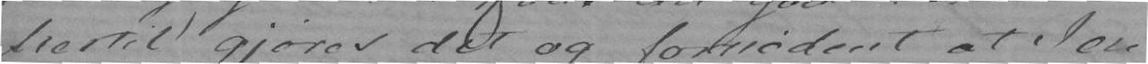hertil gjøres det og fornødent at I er
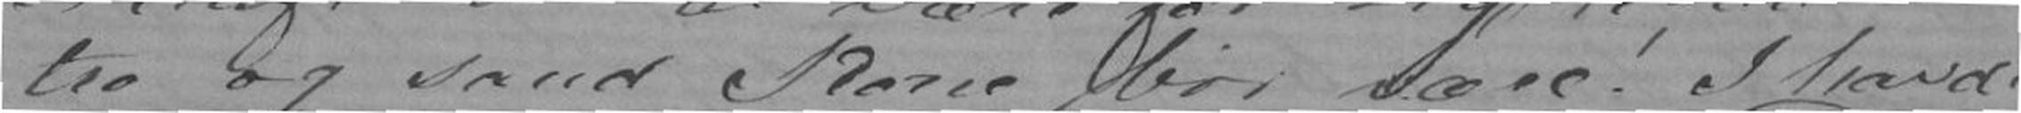tro og sand Kone bør være. I havde
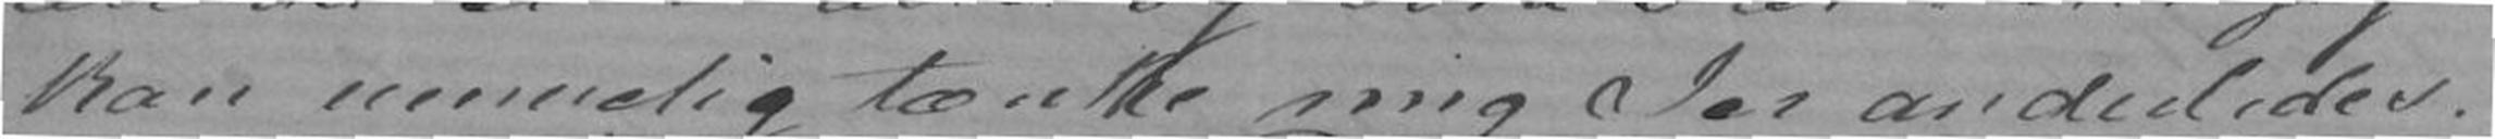kan umuelig tænke mig Jer anderledes.
